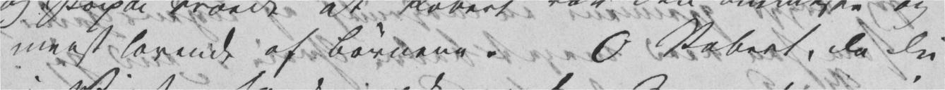meest lovende af Børnene. O Robert, da Du
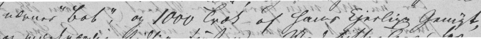nævnes «Bob», og 1000 Træk af hans kjerlige Gemyt,
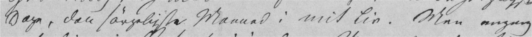Dage, den sørgeligste Maaned i mit Liv. Men engang
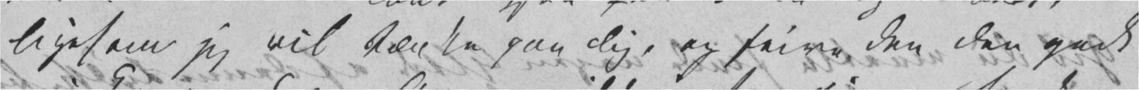ligesom jeg vil tænke paa Dig, og feire den dengodt
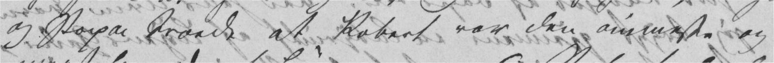og Papa troede at Robert var den ømmeste og
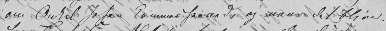om Onkel Johan kommer herned, og naar det bliver –
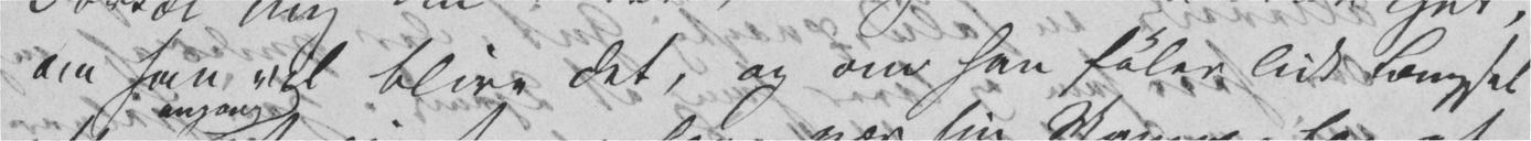om han vil blive det, og om han føler lidt Længsel
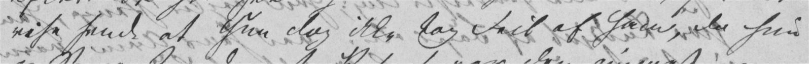vise hende at hun dog ikke tog Feil af ham, da hun
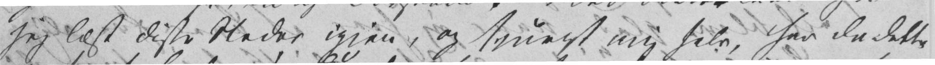jeg læst disse Steder igjen, og spurgt mig selv, har da dette
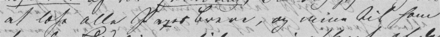at læse alle Papas Breve, og mine til ham
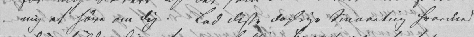mig at høre om Dig. Lad disse daglige Smaaeting hvormed
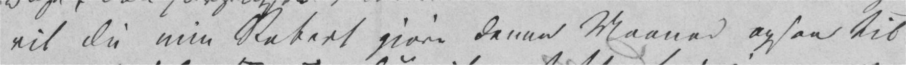vil Du min Robert gjøre denne Maaned ogsaa til
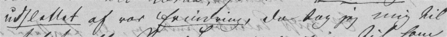udslettet af vor Erindring, da tog jeg mig til
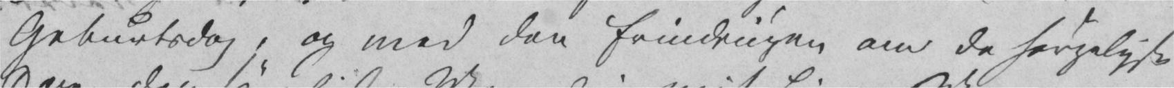Geburtsdag, og med den Erindringen om de sørgeligste
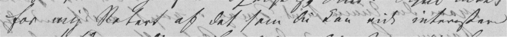for mig Robert af det som Du kan vide interesserer
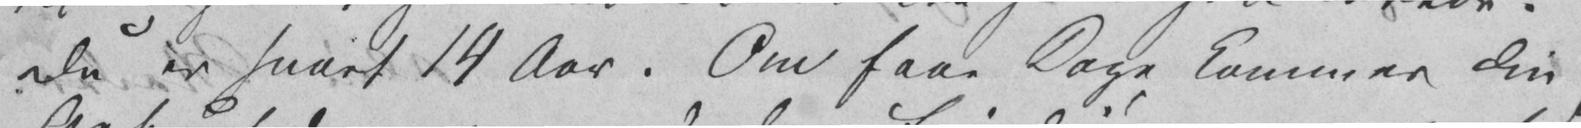Du er snart 14 Aar. Om faae Dage kommer Din
